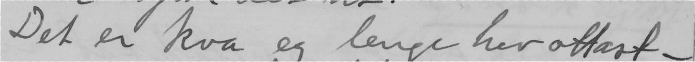Det er kva eg lenge hev ottast –
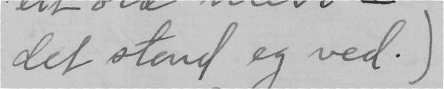det stend eg ved.
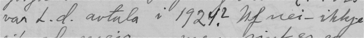var L.d. avtala i 1924? Uf nei – ikkje
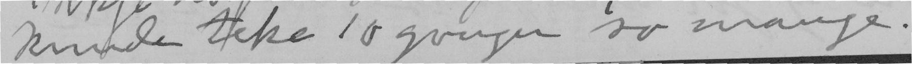kunde teke 10 gonger so mange.
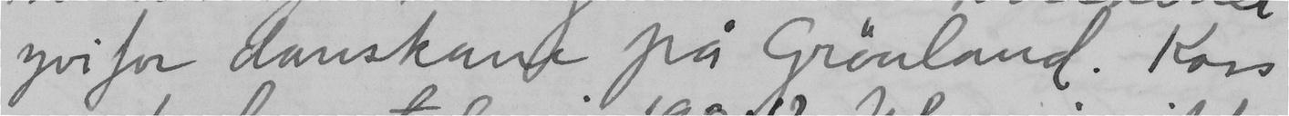yvifor danskane på Grönland. Koss
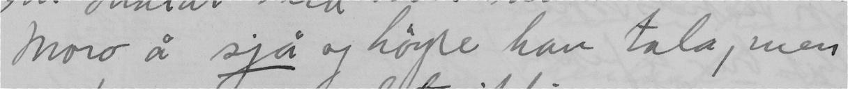Moro å sjå og höyre han tala, men
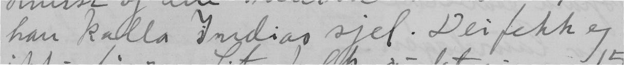han kalla Indias sjel. Dei fikk eg
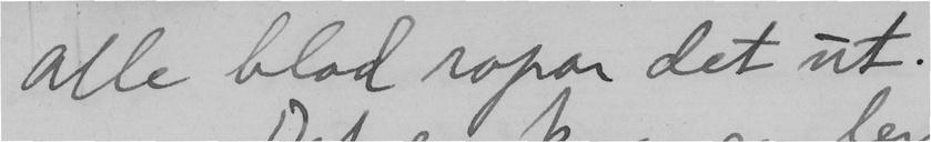Alle blad ropar det ut.
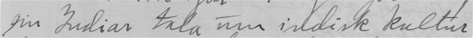ein Indiar tala um indisk kultur.
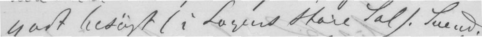godt besøgt (i Logens store Sal). Svend-
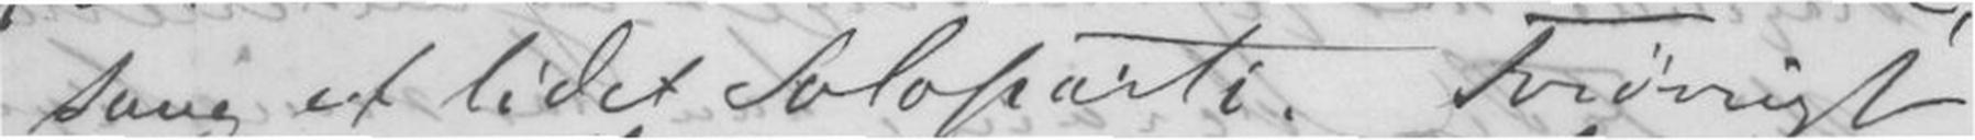sang et lidet Soloparti. Forøvrigt
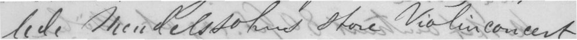lede Mendelssohns store Violinconcert
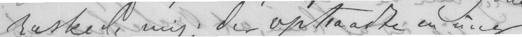raskede mig, der optraadte en ung
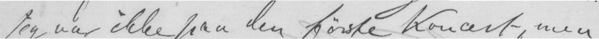Jeg var ikke paa den første Koncert, men
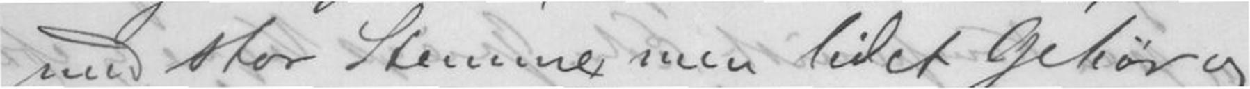med stor Stemme, men lidet Gehør og
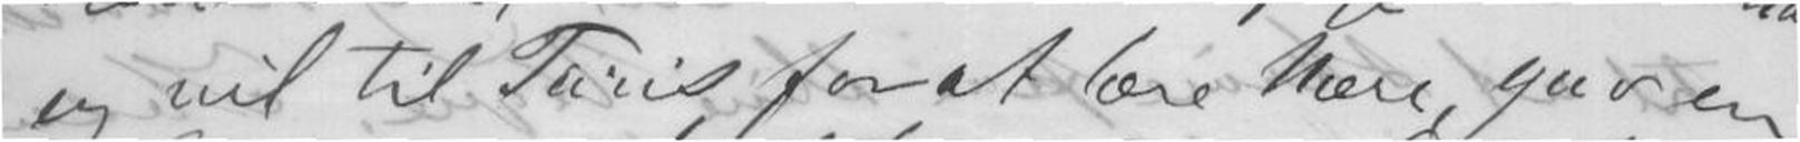og vil til Paris for at lære Mere, gav en
